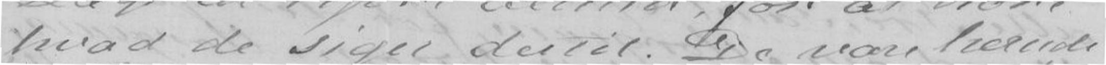hvad de siger dertil. De være herude
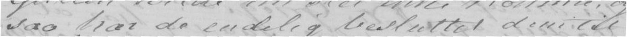saa har de endelig besluttet dem til
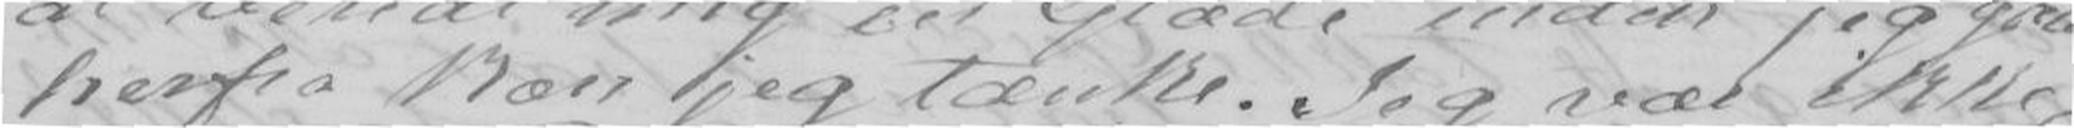herfra kan jeg tænke. Jeg var ikke
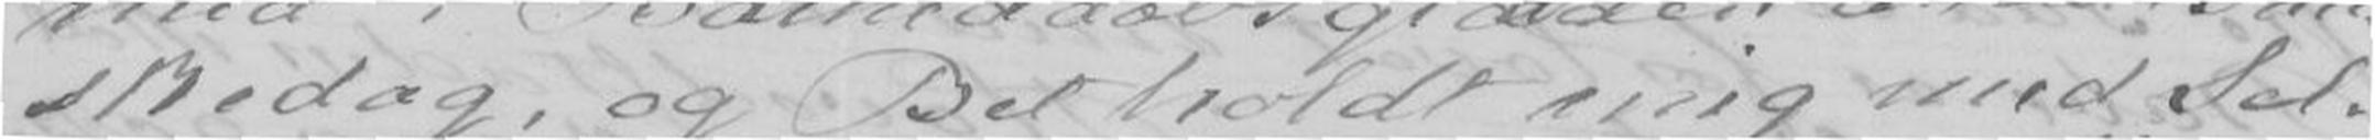skedag, og Bet holdt mig med Sel-
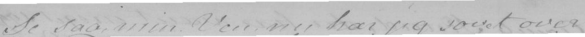Se saa min Ven nu har jeg sovet over
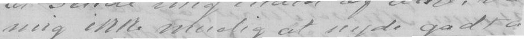mig ikke muelig at nyde godt
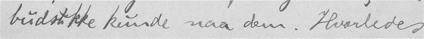budstikke kunde naa dem. Hvorledes
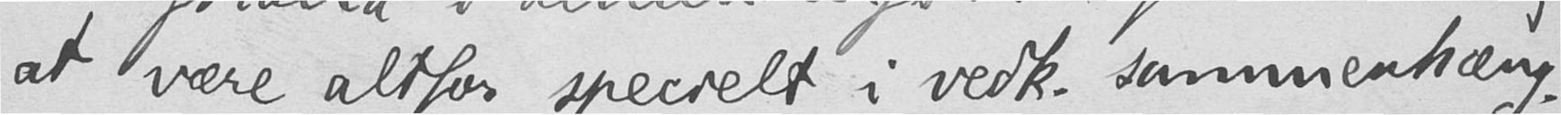at være altfor specielt i vedk. sammenhæng.
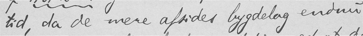tid, da de mere afsides bygdelag endnu
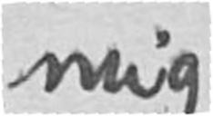mig
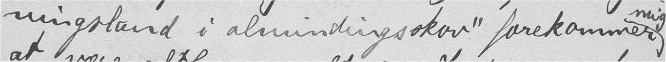ningsland i almindingsskov" forekommer
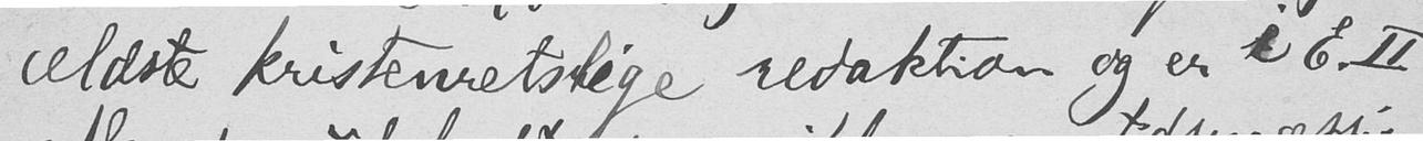ældste kristenretslige redaktion og er i E. II
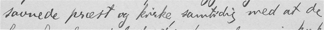savnede præst og kirke, samtidig med at de
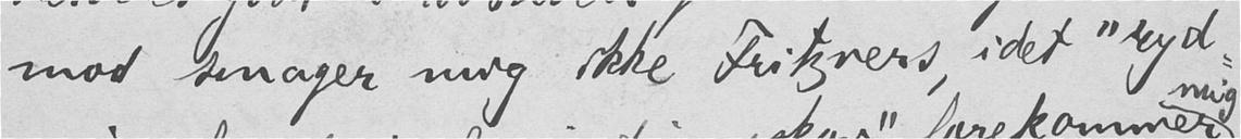mod smager mig ikke Fritzners, idet "ryd-
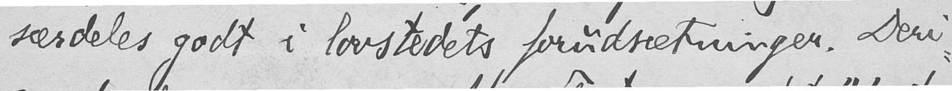særdeles godt i lovstedets forudsætninger. Deri-
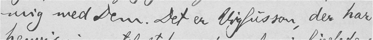mig med Dem. Det er Vigfusson, der har
